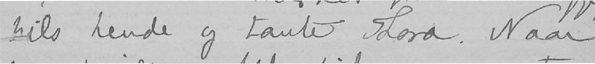hils hende og tante Thora. Naar
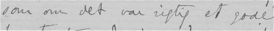som om det var rigtig et gode
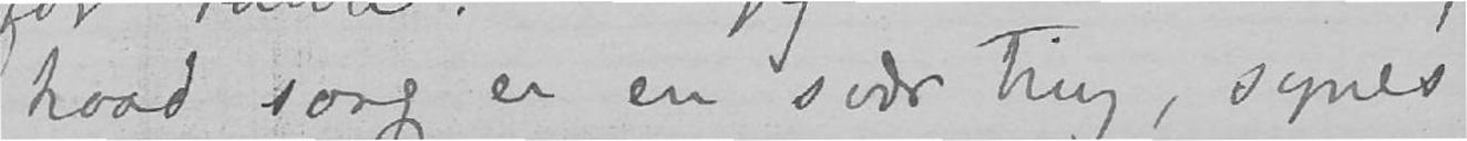hvad sorg er en svær ting, synes
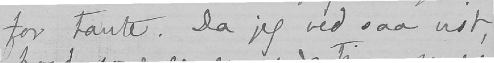for tante. Da jeg ved saa vist,
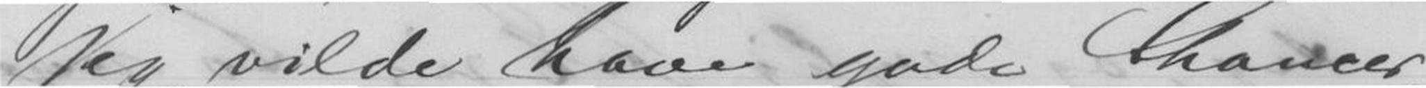jeg vilde have gode Chancer,
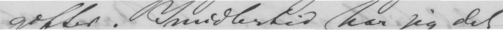gifter. Imidlertid har jeg det
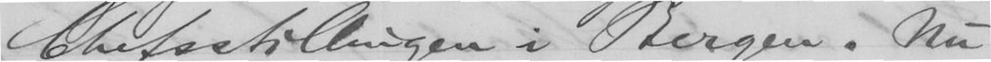Chefsstillingen i Bergen. Nu -
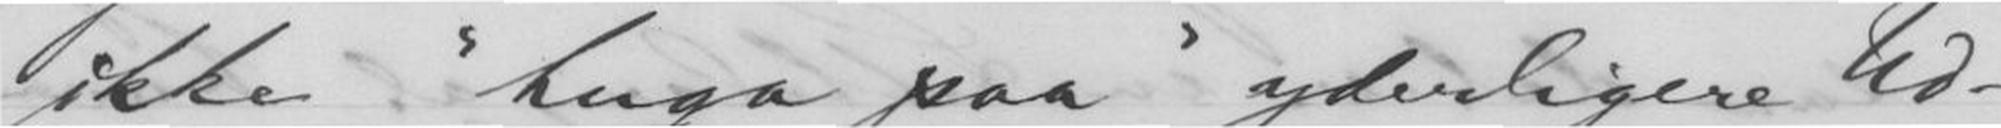ikke "huga paa" yderligere Ud-
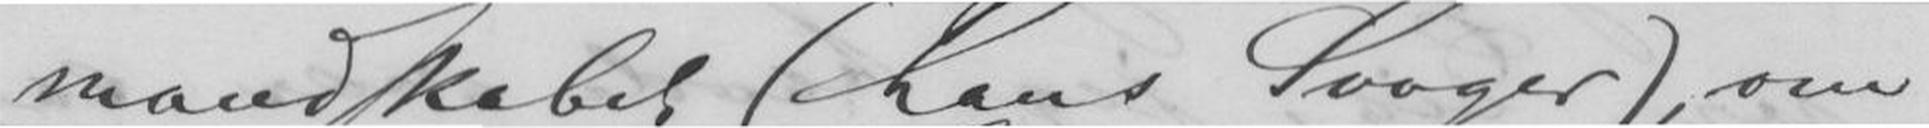mandskabet (hans Svoger), som
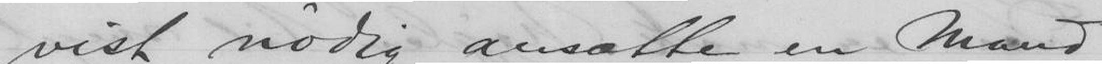vist nødig ansætte en Mand
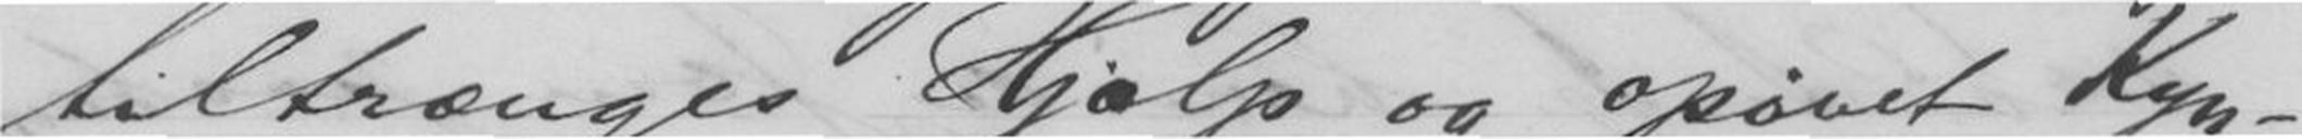tiltrænges Hjælp og opøvet Kyn-
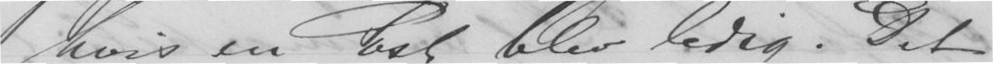hvis en Post blir ledig. Det
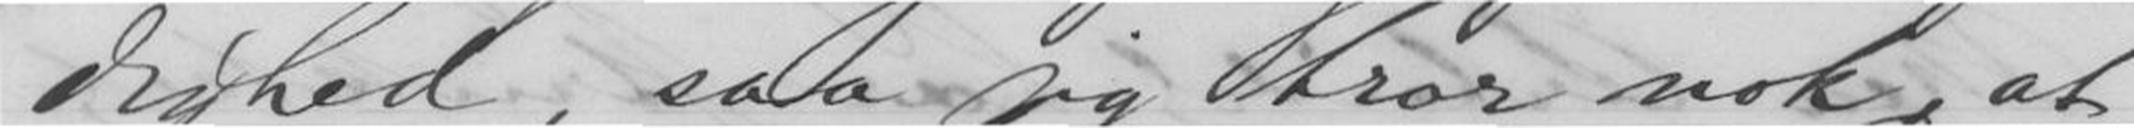dighed, saa jeg tror nok, at
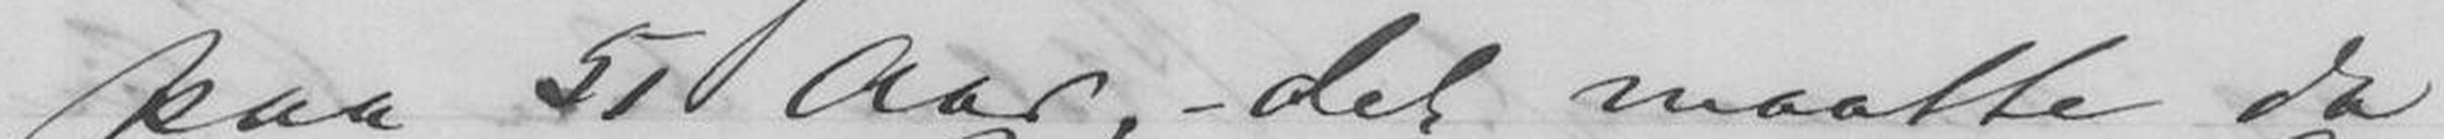paa 51 Aar, - det maatte da
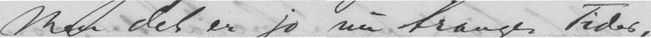Men det er jo nu trange Tider,
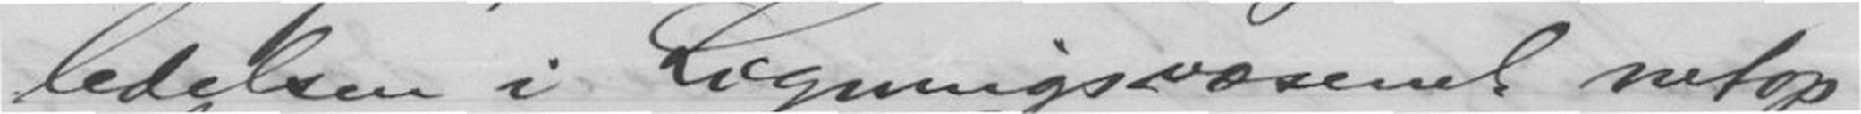ledelsen i Ligningsvæsenet netop
